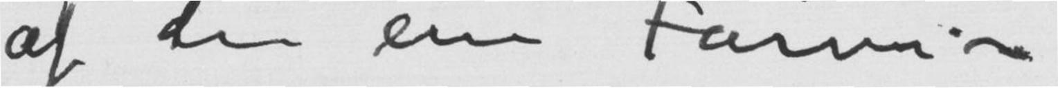af denne ene Farve –
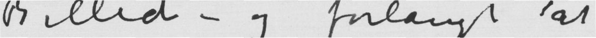Billede – og forlangt at
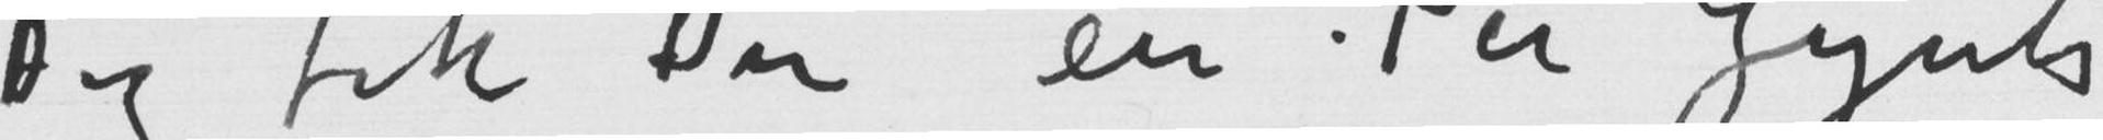Dig fik Du en Per Gynts
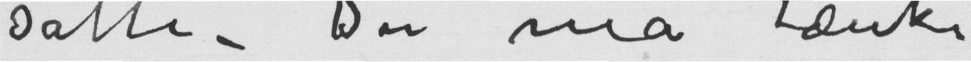satte – Du må tænke
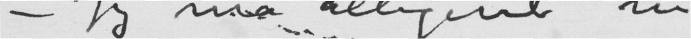– Jeg må alligevel nu
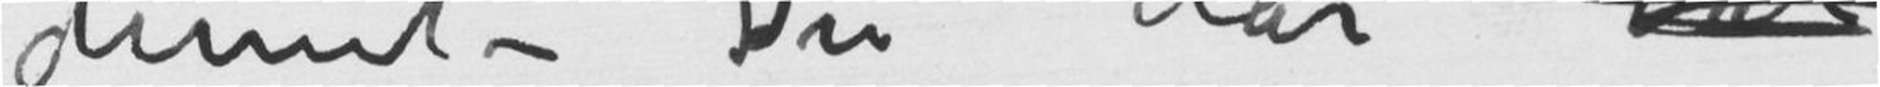dumt – Du har
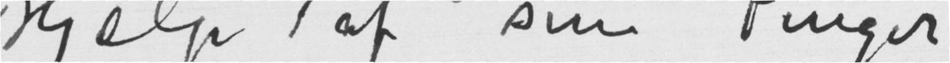Hjælp af sine Penger
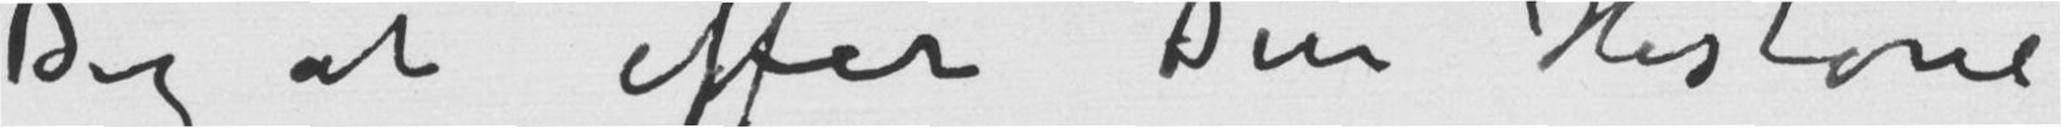Dig at efter Din Historie
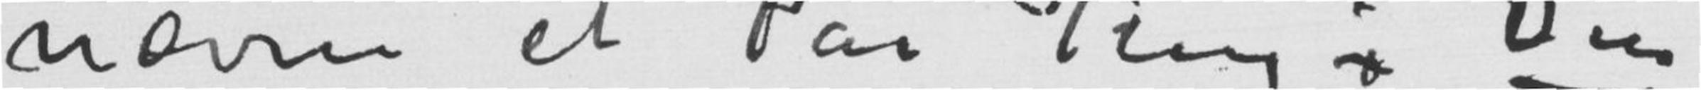nævne et par Ting :– Din
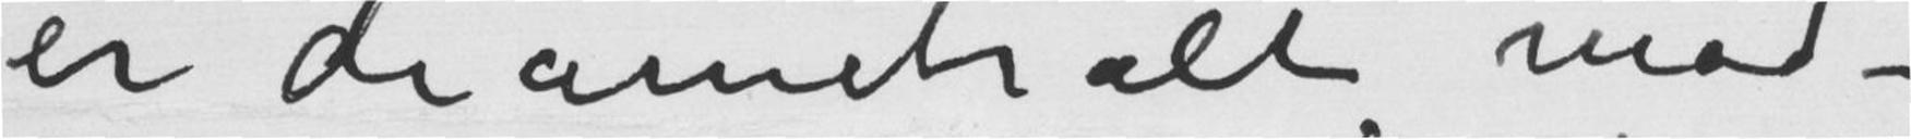er diametralt mod-
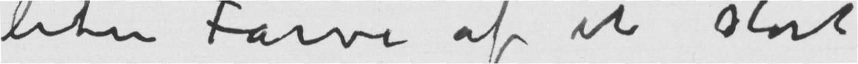liten Farve af et stort
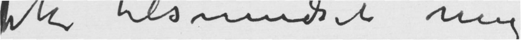fik tilsmudset mig
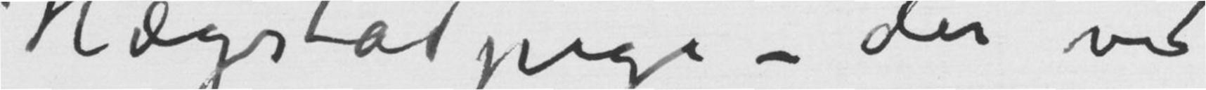Hægstadpige – der ved
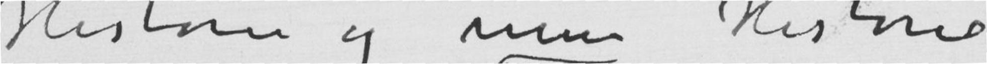Historie og min Historie
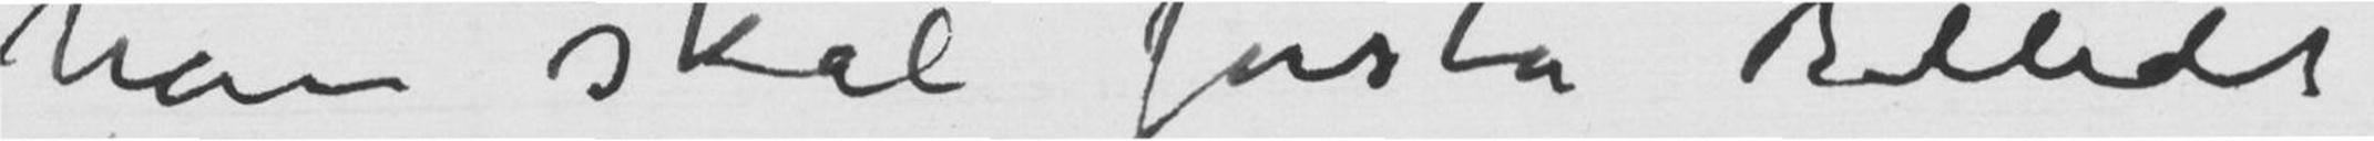han skal forstå Billedet
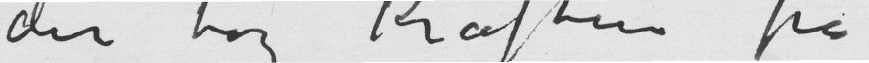der tog Kraften fra
